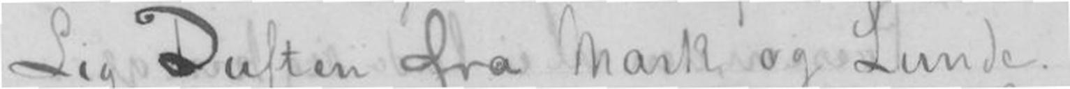Lig Duften fra Mark og Lunde
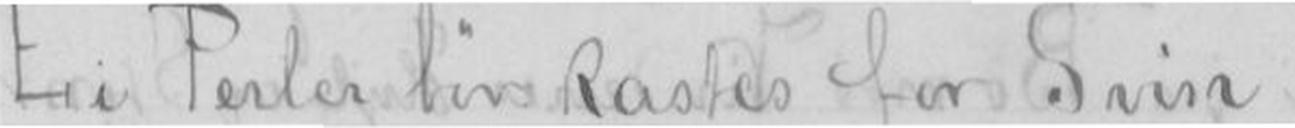Ei Perler bør kastes for Svin
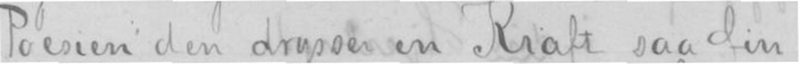Poesien den drysser en Kraft saa fin
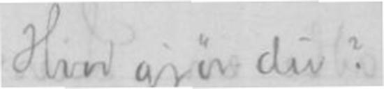Hvad gjør du?
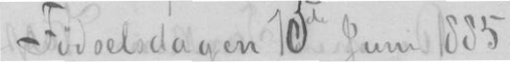Fødselsdagen 15de Juni 1885.
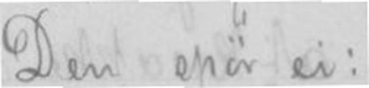Den spør ei:
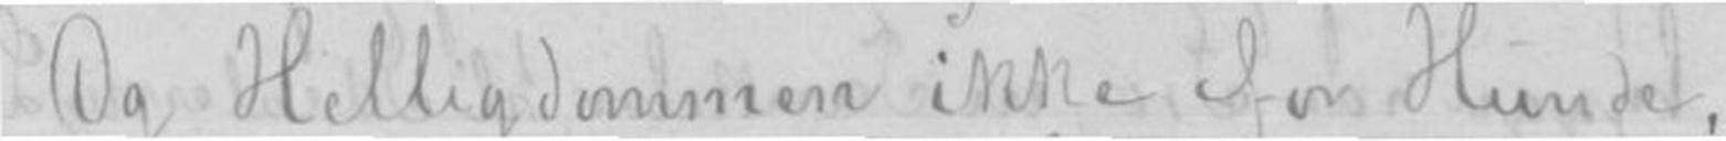Og Helligdommen ikke for Hunde,
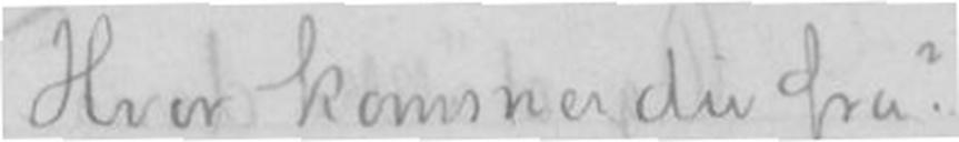Hvor kommer du fra?
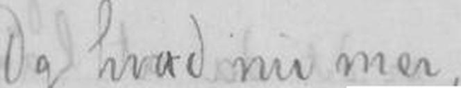Og hvad nu mer,
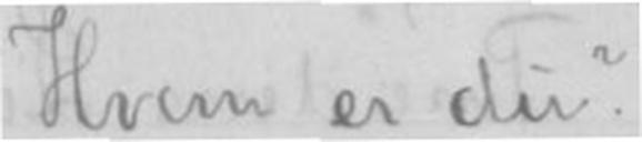Hvem er du?
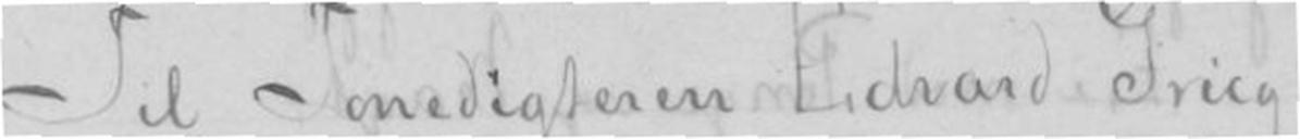Til Tonedigteren Edvard Grieg
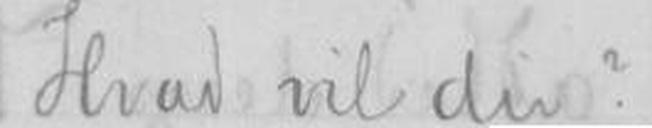Hvad vil du?
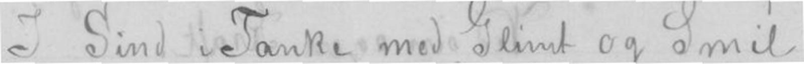I Sind i Tanke med Glimt og Smil
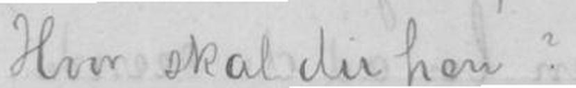Hvor skal du hen?
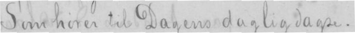Som hører til Dagens daglig dagse.
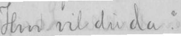Hvad vil du da?
